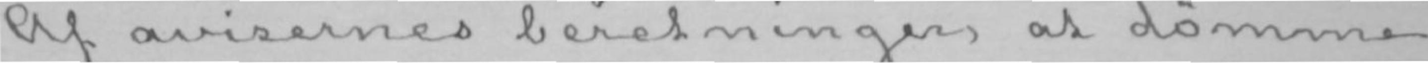Af avisernes beretninger at dømme
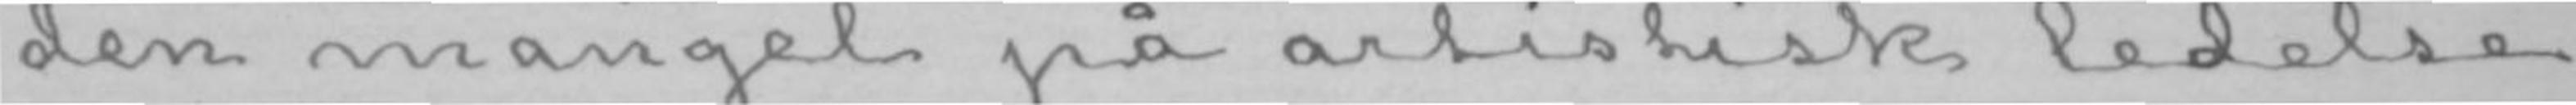den mangel på artistisk ledelse,
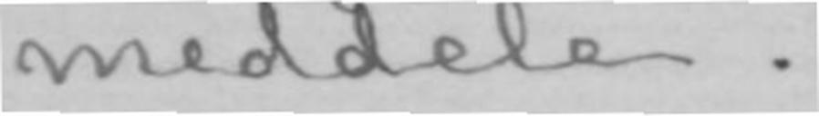meddele.
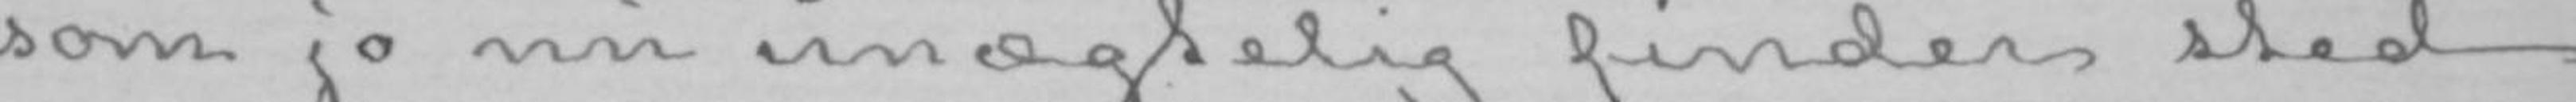som jo nu unægtelig finder sted
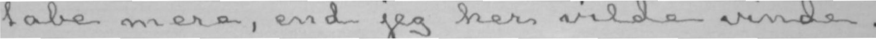tabe mere, end jeg her vilde vinde.
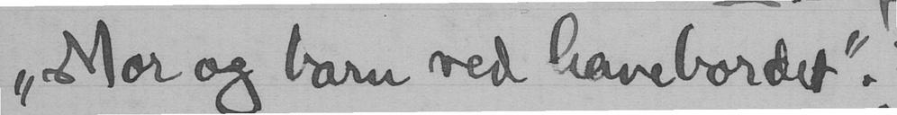"Mor og barn ved havebordet".
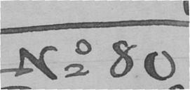No 80
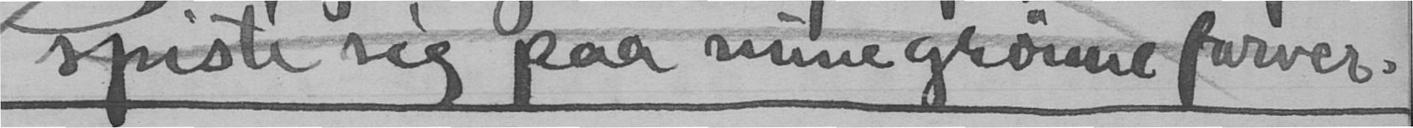spiste sig paa mine grønne farver.
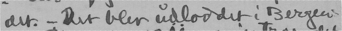det. - Det blev udloddet i Bergen-
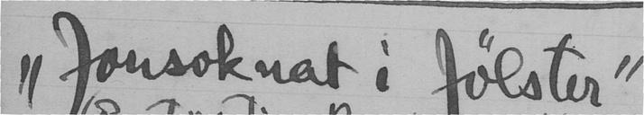"Jonsoknat i Jølster"
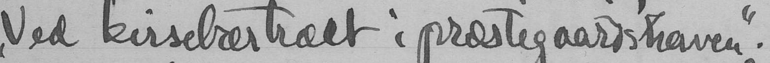"Ved kirsebærtræet i præstegaardshaven". Eier: ukjendt. Billedet
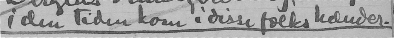i den tiden kom i disse folks hænder.
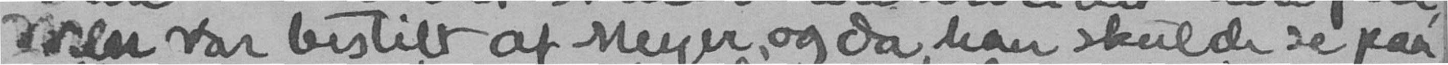men var bestilt af Meyer, og da han skulde se paa
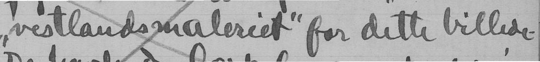"vestlandsmaleriet" for dette billede.
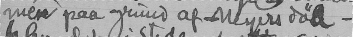men paa grund af Meyers død -
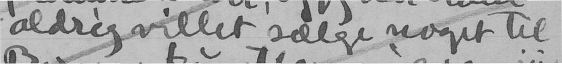aldrig villet sælge noget til
